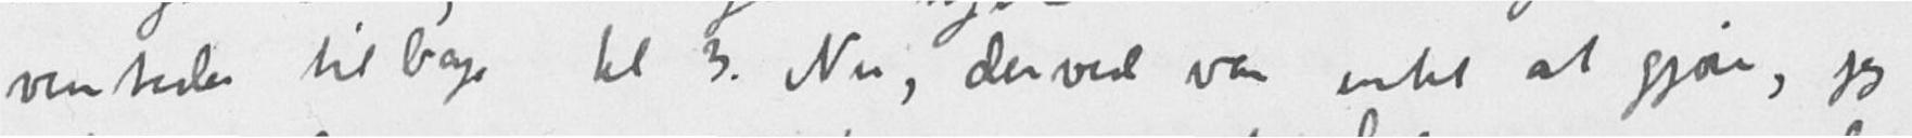ventede tilbage kl 3. Nu, derved var intet at gjøre, jeg
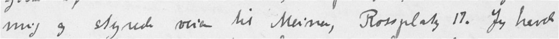mig og styrede veien til Meiner, Rossplatz 17. Jeg havde
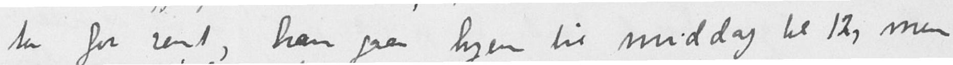ter for sent, han gaar hjem til middag kl 12, men
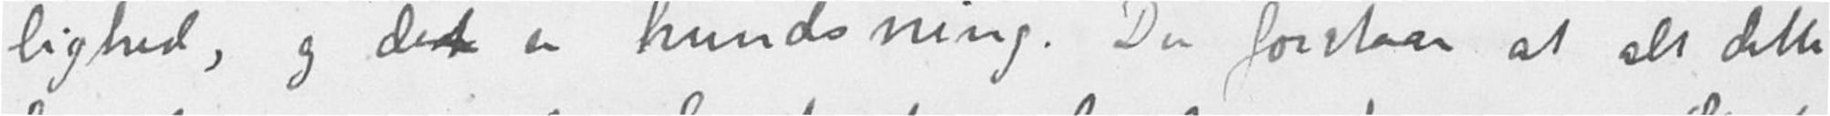lighed, og det er hundsning. Du forstaar at alt dette
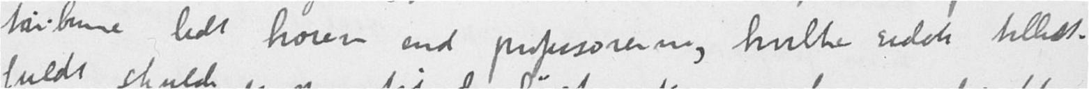tribune lidt høiere end professorerne, hvilke sidder tillidt-
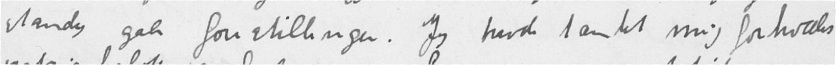stændig gale forestillinger. Jeg havde tænkt mig forhoder
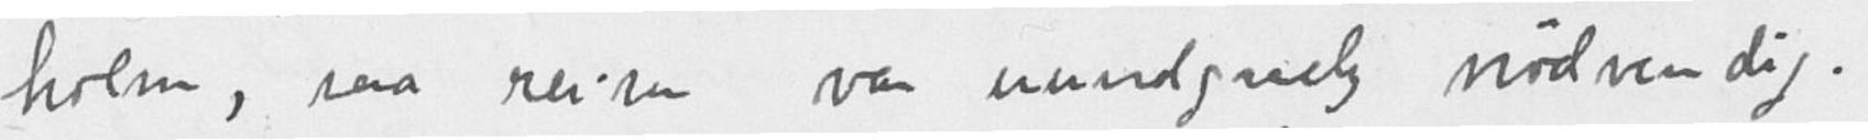holm, saa reisen var uundgaaelig nødvendig.
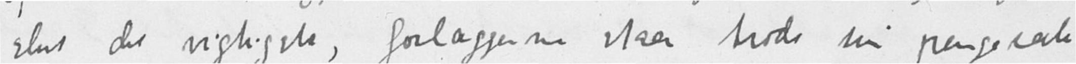slut det vigtigste, forlæggerne staar trods sin pengesæk
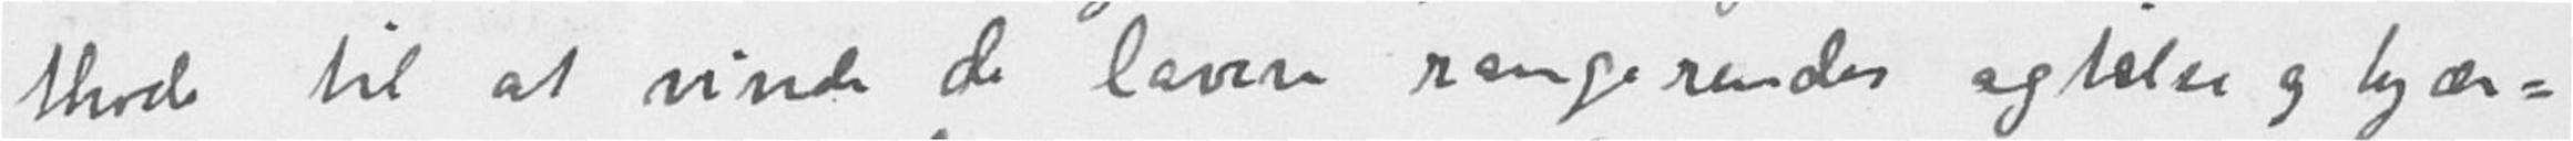thode til at vinde de lavere rangerendes agtelse og kjær-
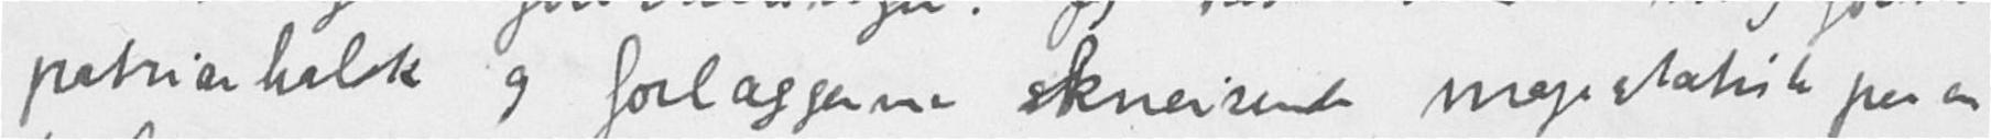patriarkalsk og forlæggerne kneisende majestætiske paa en
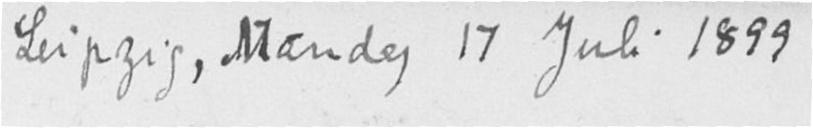Leipzig, Mandag 17 Juli 1899
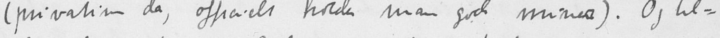(privatisen da, officielt holder man gode miner). Og til-
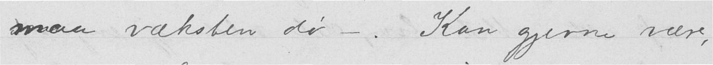maa væksten dø -. Kan gjerne være,
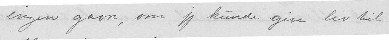ingen gavn, om jeg kunde give liv til
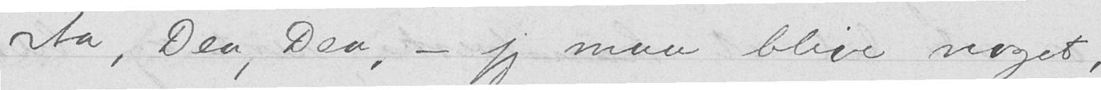Aa, Dea, Dea, - jeg maa blive noget,
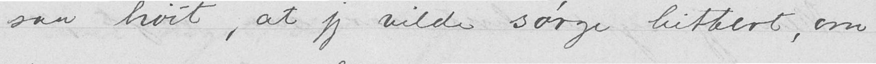saa høit, at jeg vilde sørge bittert, om
